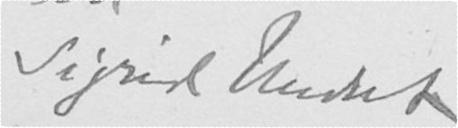Sigrid Undset
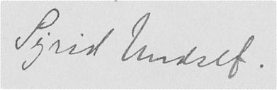Sigrid Undset.
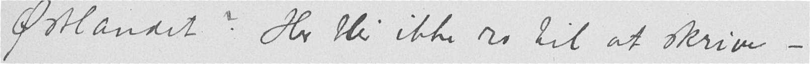Østlandet? Her blir ikke ro til at skrive –
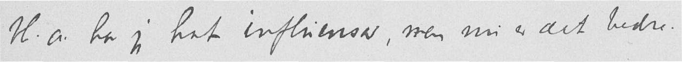bl.a. har jeg hat influensa, men nu er det bedre.
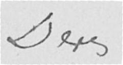Deres
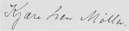Kjære herr Møller,
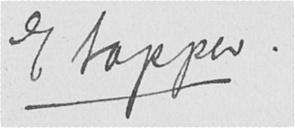Etapper.
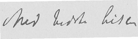Med bedste hilsen
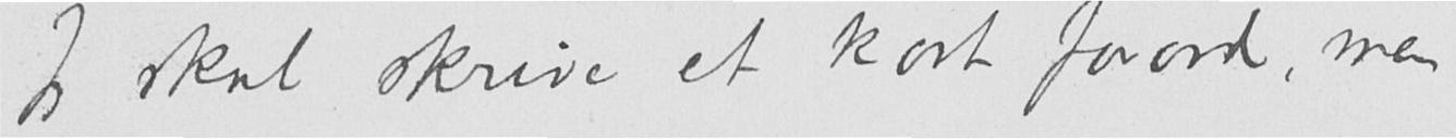Jeg skal skrive et kort forord, men
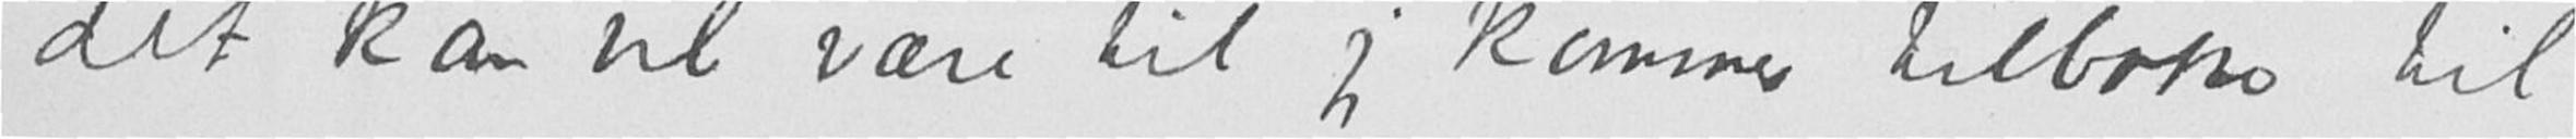det kan vel være til jeg kommer tilbake til
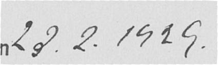22.2.1929.
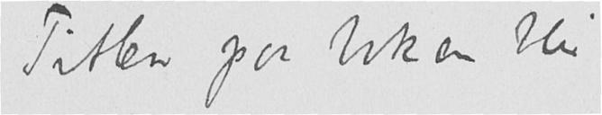Titlen paa boken blir
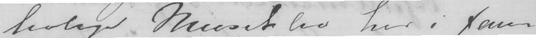livlige Musikliv her i Xania
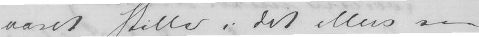væet stille i det ellers saa
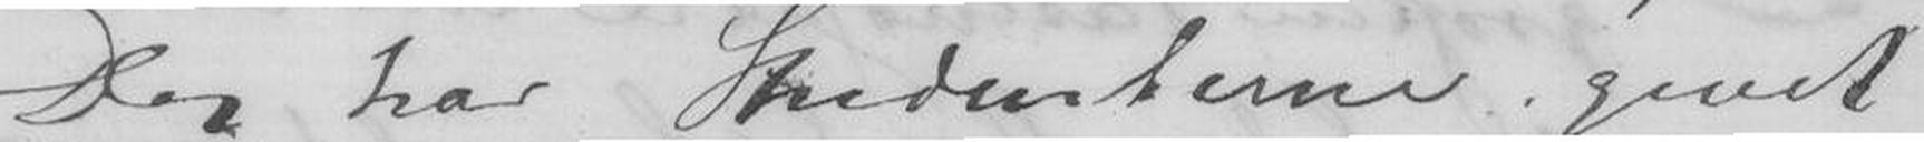Dog har Studenterne givet
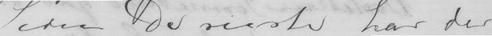Siden De reiste har der
